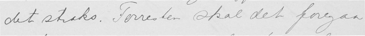det straks. Forresten skal det foregaa
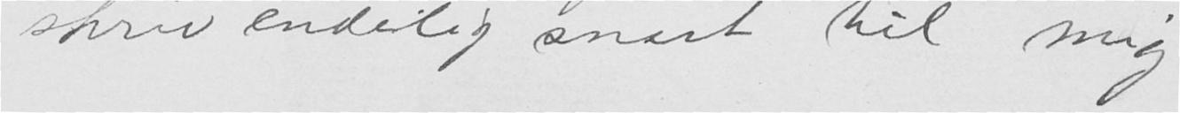skriv endelig snart til mig
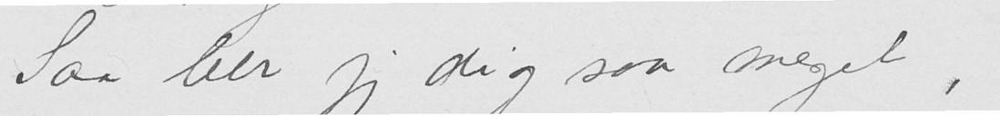Saa ber jeg dig saa meget,
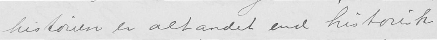historien er alt andet end historisk
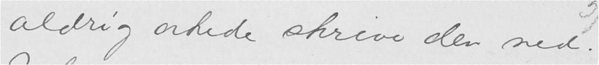aldrig orkede skrive den ned.
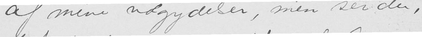af mine udgydelse, men ser du,
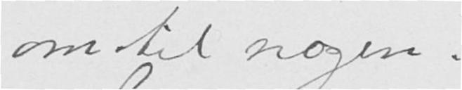om til nogen.
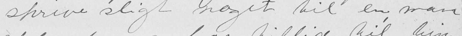skrive sligt noget til en man
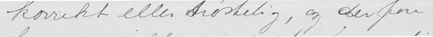korrekt eller trøstelig, og der fore-
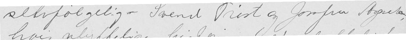selvfølgelig – Svend Trøst og Jomfru Agnete
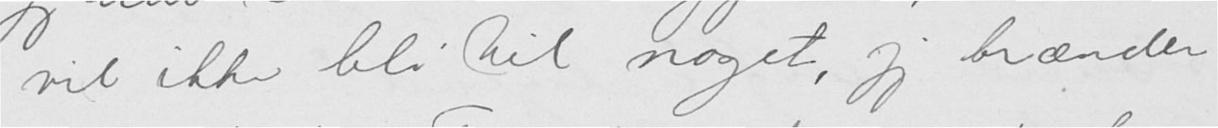vil ikke bli til noget, jeg brænder
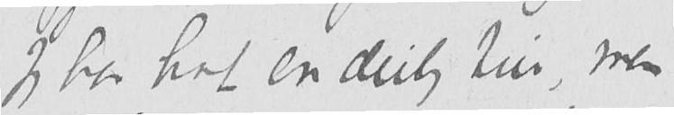Jeg har hat en deilig tur, men
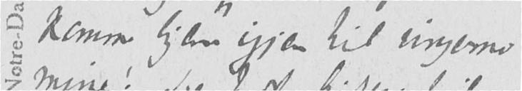komme hjem igjen til ungerne
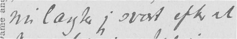nu længter jeg svært efter at
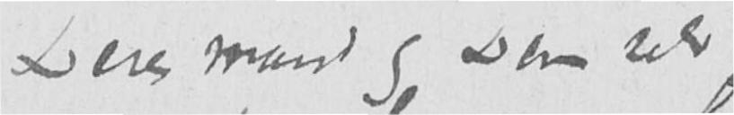Deres mand og Dem selv
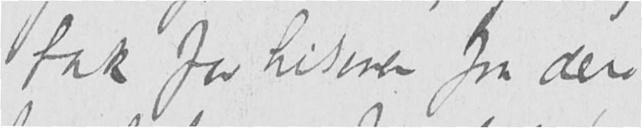tak for hilsener fra dere
